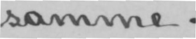samme.
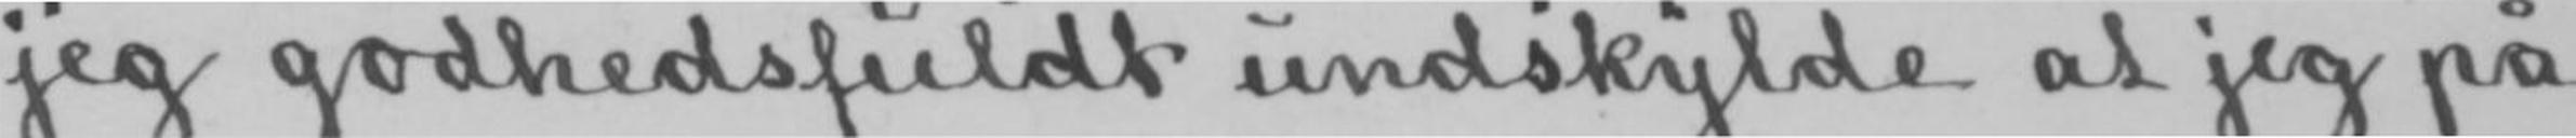jeg godhedsfuldt undskylde at jeg på
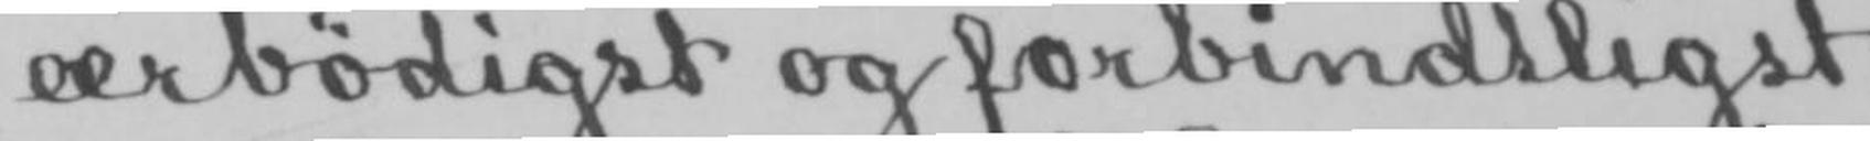ærbødigst og forbindtligst
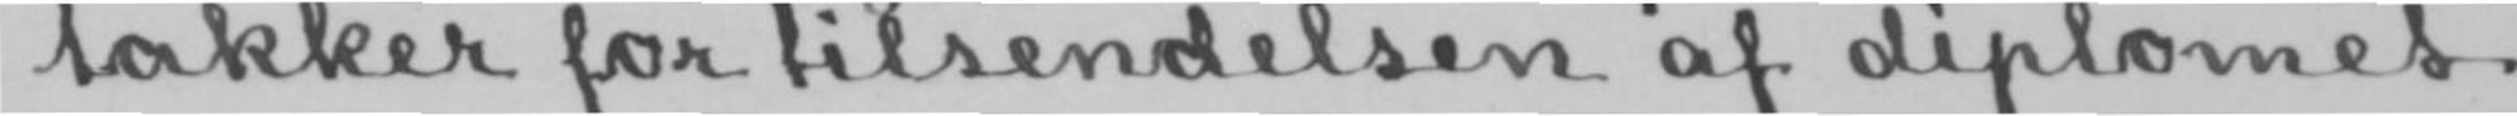takker for tilsendelsen af diplomet
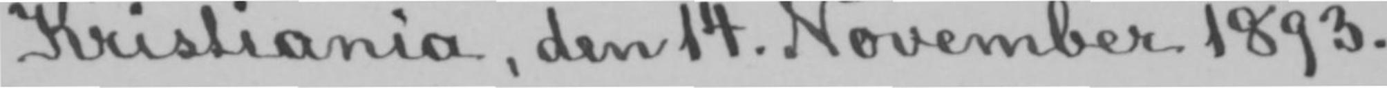Kristiania, den 14. November 1893.
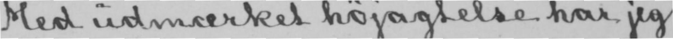Med udmærket højagtelse har jeg
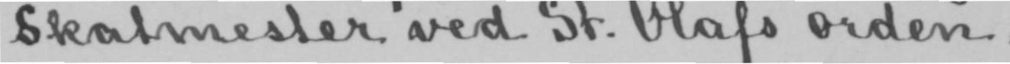Skatmester ved St. Olafs orden.
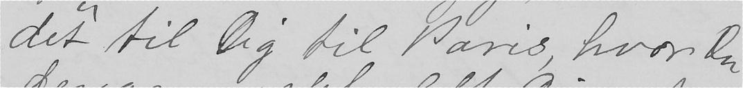det til Dig til Paris, hvor Du
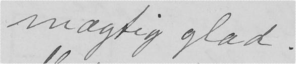mægtig glad.
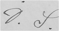D.S.
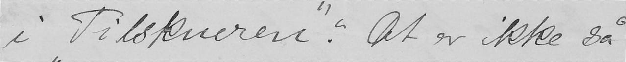i "Tilskueren". Det er ikke så
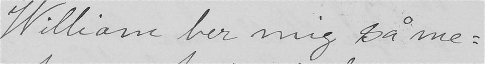William ber mig så me-
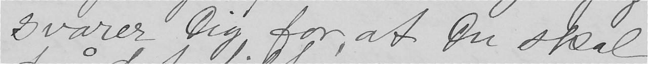svarer Dig for, at Du skal
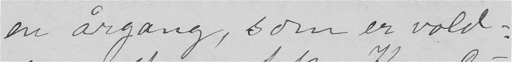en årgang, som er vold-
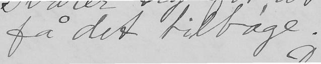få det tilbage.
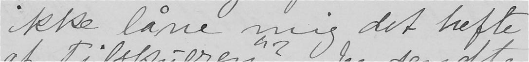ikke låne mig det hefte
